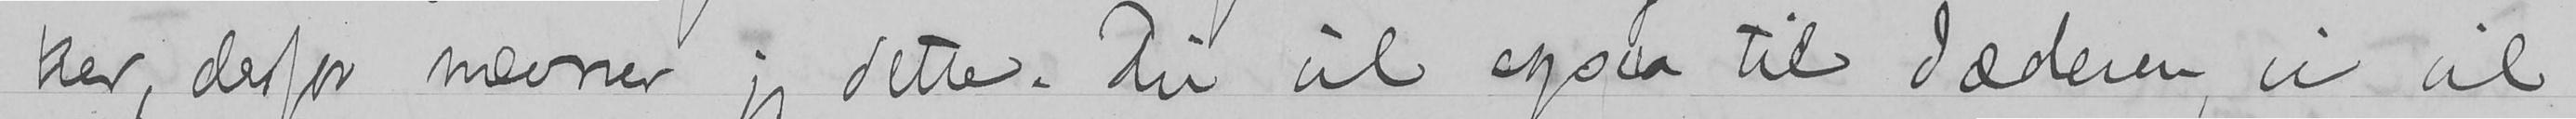ker, derfor nævner jeg dette. Du vil ogsaa til Jæderen, vi vil
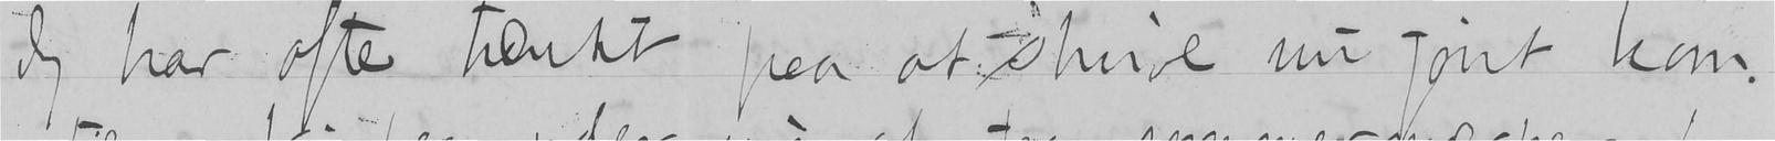Jeg har ofte tænkt paa at skrive nu først kom-
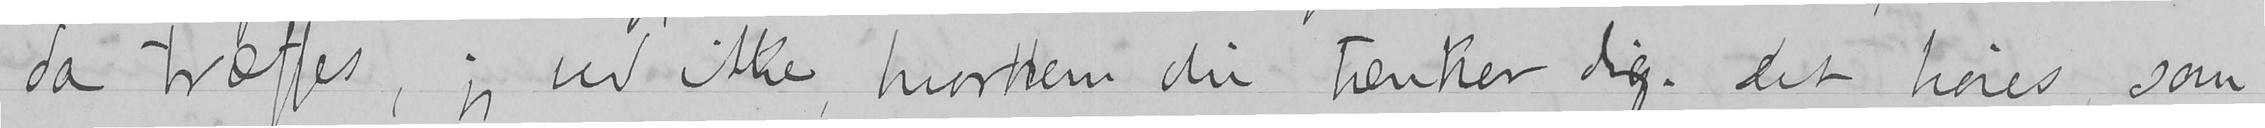da træffes, jeg ved ikke hvorhen du tænker dig. Det høres, som
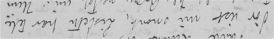dør vist nu snart, Lisbeth har tele-
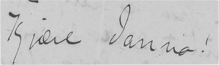Kjære Janna!
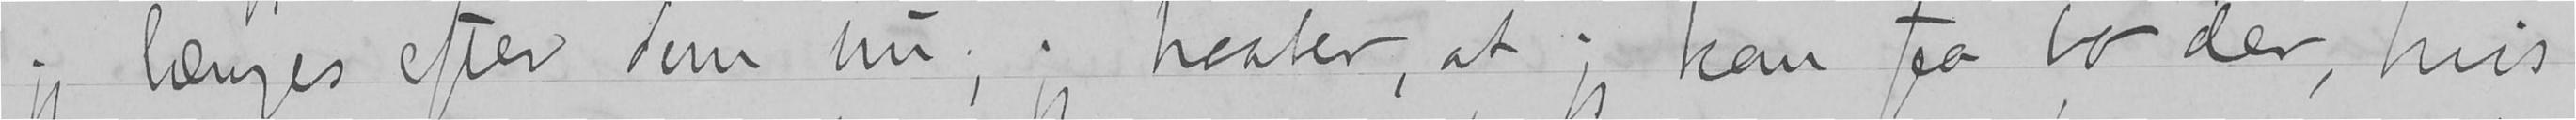jeg længes efter dem nu, jeg haaber, at jeg kan faa bo der, hvis
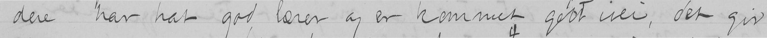dere har hat god lærer og er kommet godt ivei, det gir
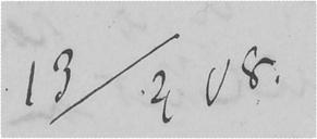13/4 08.
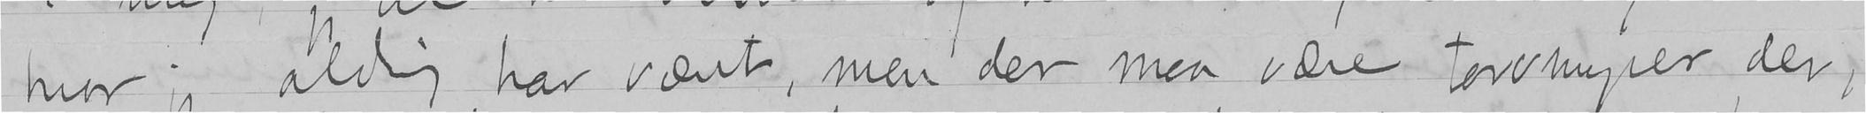hvor jeg aldrig har været, men der maa være torvmyrer der,
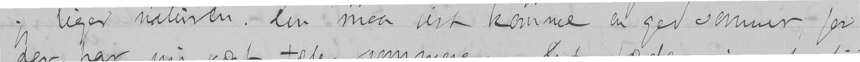jeg liger naturen. Der maa vist komme en god sommer, for
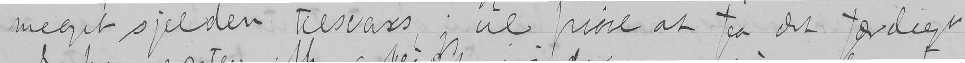meget sjelden tilsvars, jeg vil prøve at faa det færdigt.
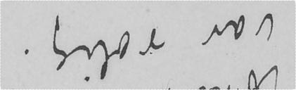var rolig.
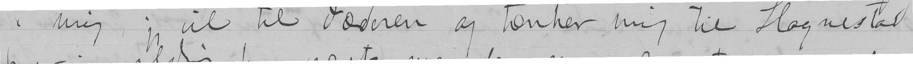i mig, jeg vil til Jæderen og tænker mig til Hognestad
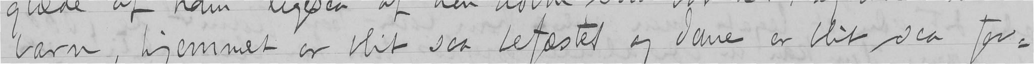barn, hjemmet er blit saa befæstet og Jane er blit saa for-
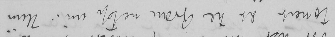fonert det til Gram netop nu. Hun
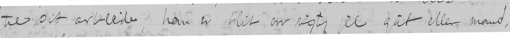til sit arbeide, han er blit en rigtig jil gut eller mand,
